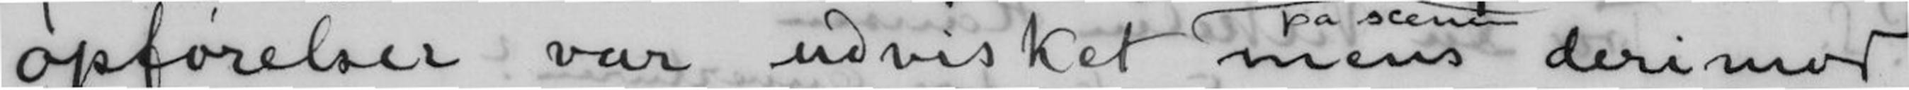opførelser var udvisket mens derimod
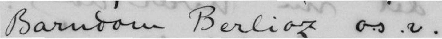Barndom Berlioz o.s.v. ----
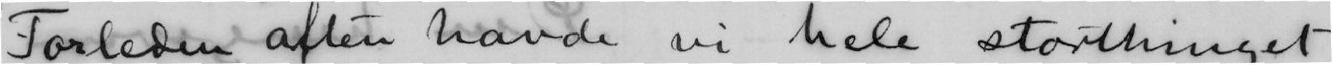Forleden aften havde vi hele storthinget
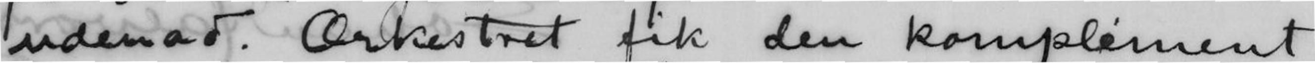udenad. Orkestret fik den kompliment
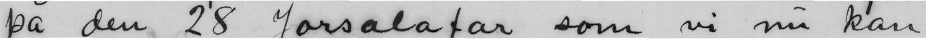på den 28 Jorsalafar som vi nu kan
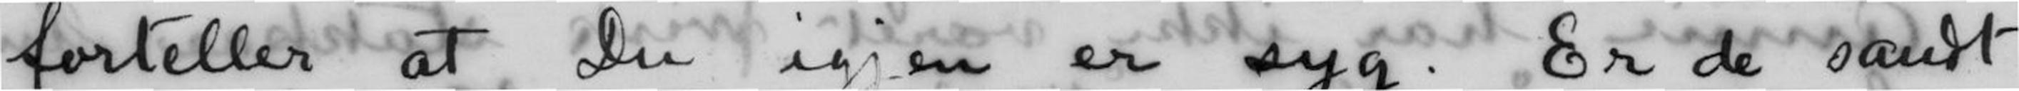forteller at Du igjen er syg. Er de sandt
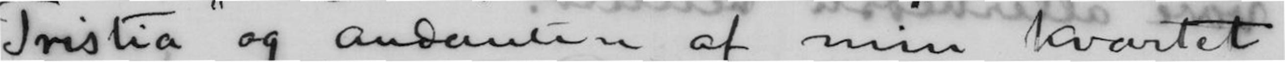Tristia og andanten af min kvartet.
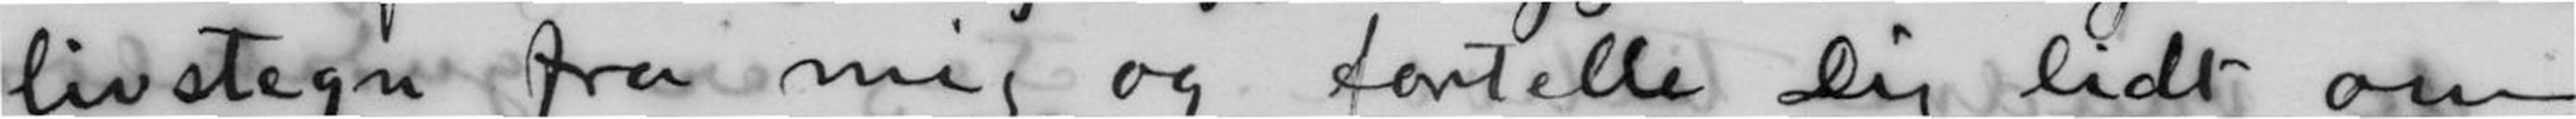livstegn fra mig og fortelle Dig lidt om
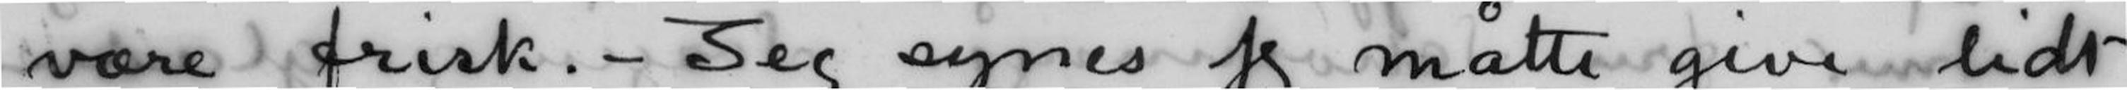være frisk. - Jeg synes jeg måtte give lidt
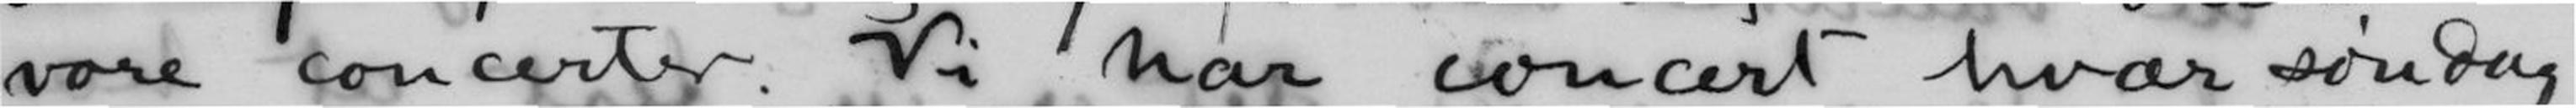vore concerter. Vi har concert hvær søndag
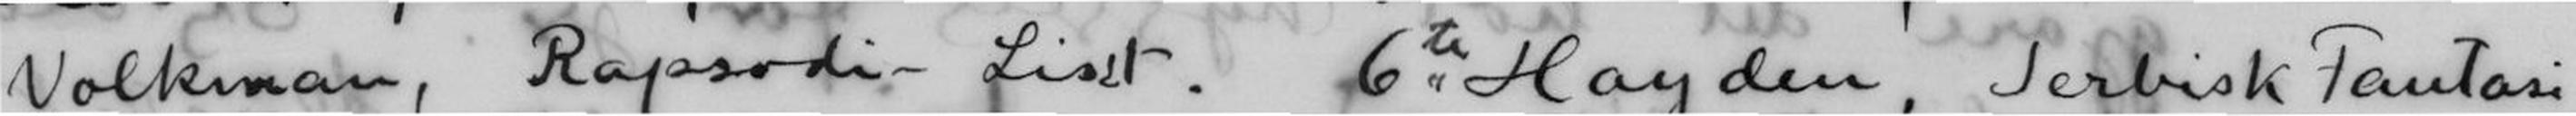Volkman, Rapsodi Liszt. 6te Hayden, Serbisk Fantasi
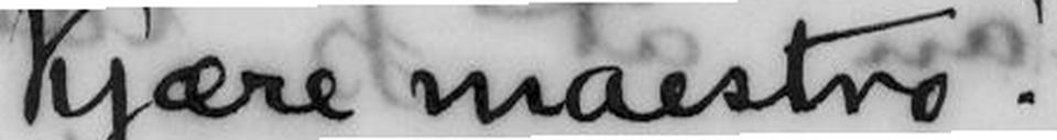Kjære maestro!
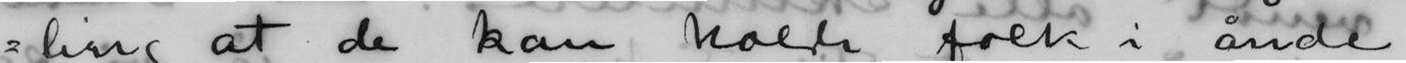ling at de kan holde folk i ånde.
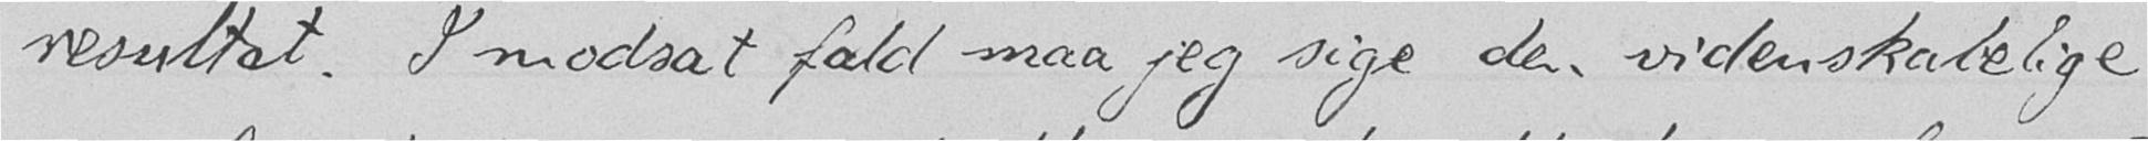resultat. I modsat fald maa jeg sige den videnskabelige
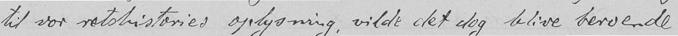til vor retshistories oplysning, vilde det dog blive beroende
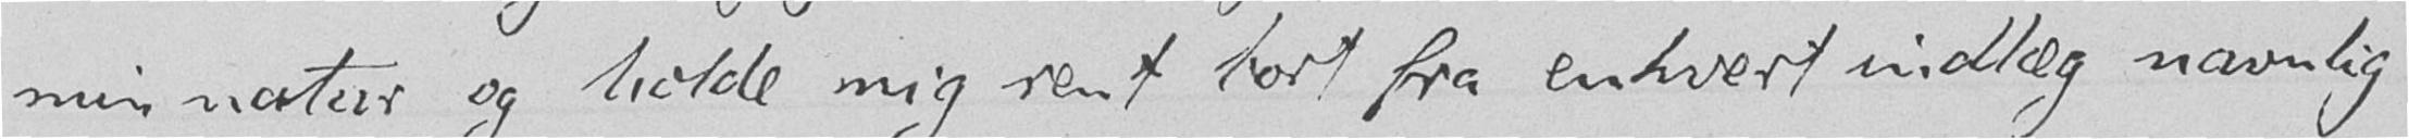min natur og holde mig rent bort fra enhvert indlæg navnlig
